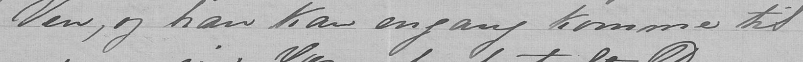Ven, og han kan engang komme til
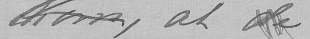kom, at de
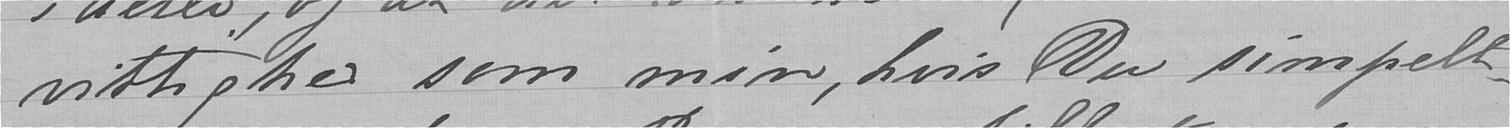vittighed som min, hvis Du simpelt
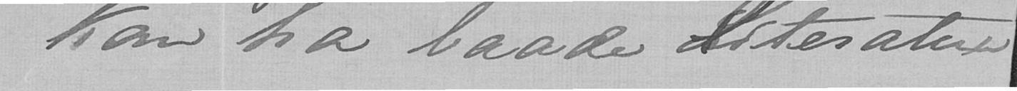kan ha baade Literatur
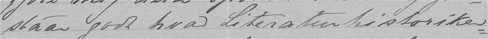staar godt hvad Literaturhistoriker-
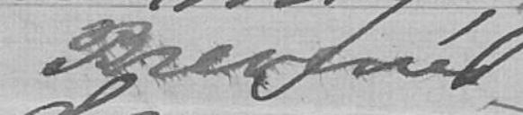Brevene
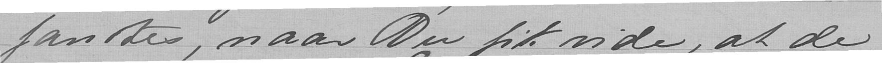fandtes, naar Du fik vide, at de
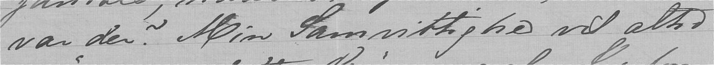var der? Min Samvittighed vil altid
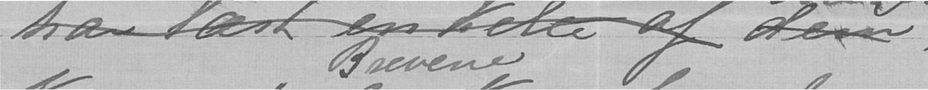har læst enkelte af dem,
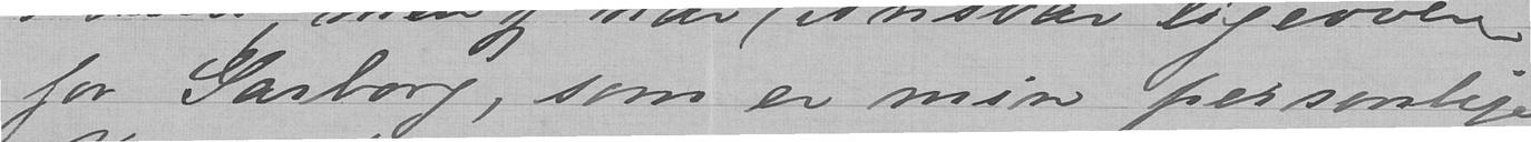for Garborg, som er min personlige
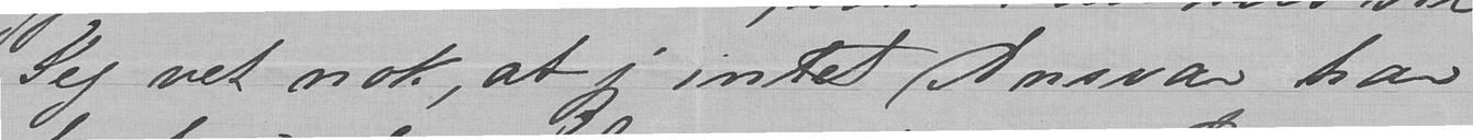Jeg vet nok, at jeg intet Ansvar har
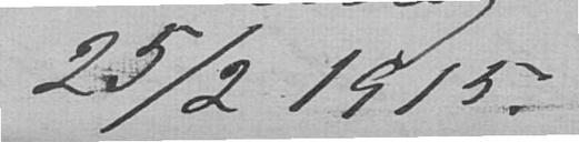25/2 1915.
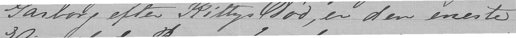Garborg efter Kittys Død, er den eneste
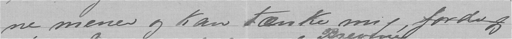ne mener og kan tænke mig, fordi jeg
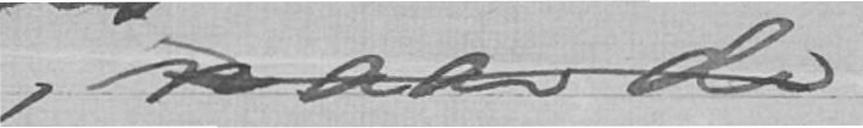naar de
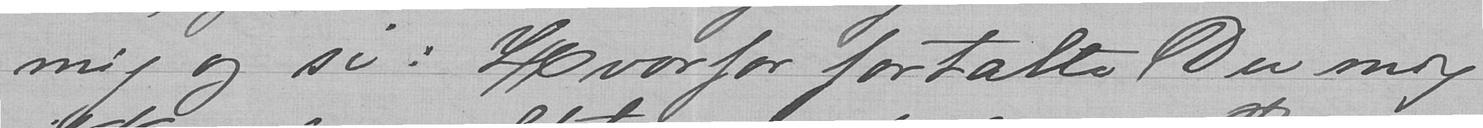mig og si: Hvorfor fortalte Du mig
